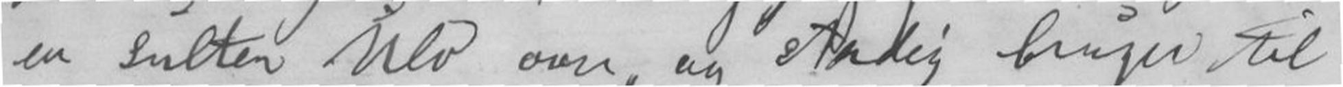en sulten Ulv over, og stadig brunger til
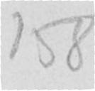158
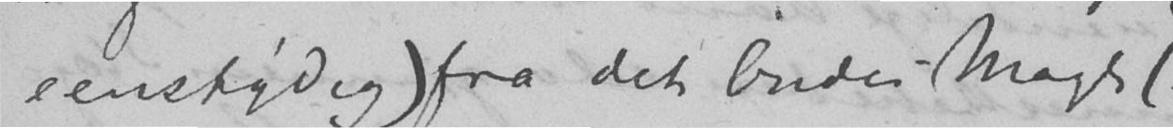eenstydig) fra det Ondes Magt
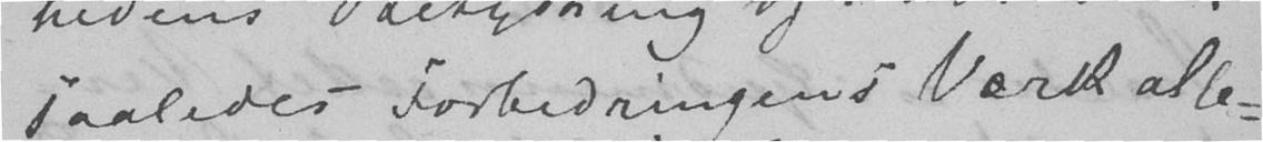saaledes Forbedringens Værk alle-
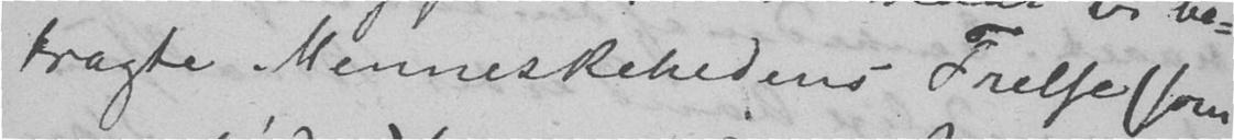tragte Menneskehedens Frelse (som
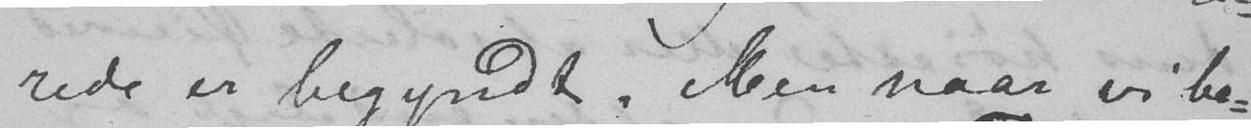rede er begyndt. Men naar vi be-
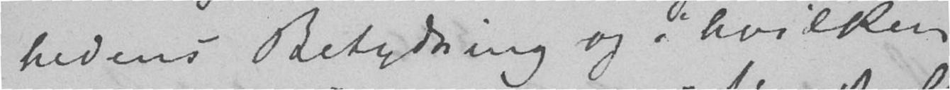hedens Betydning og i hvilken
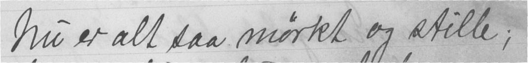Nu er alt saa mørkt og stille;
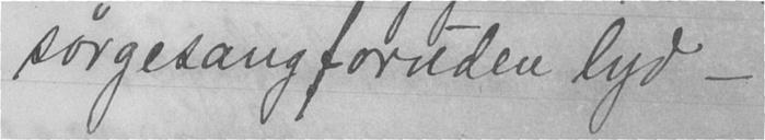sørgesang foruden lyd -
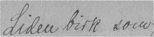Liden birk som
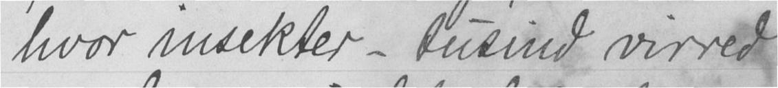hvor isekter - tusind virred
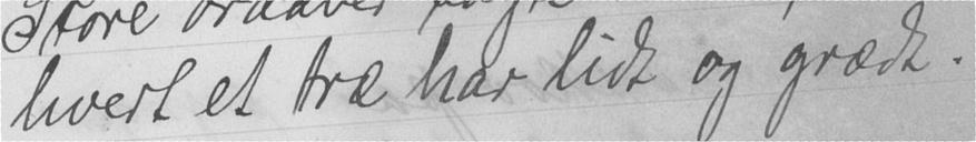hvert et træ har lidt og grædt.
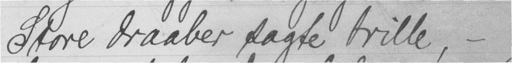Store draaber sagte trille, -
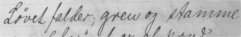Løvet falder; gren og stamme
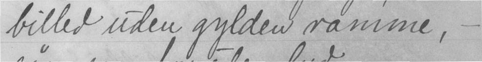billed uden gylden ramme, -
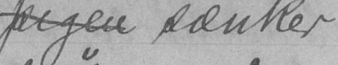pigen sænker
